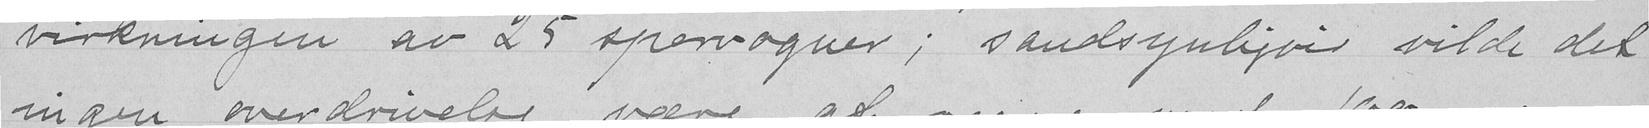virkningen av 25 spervogner; sandsynligvis vilde det
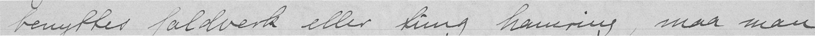benyttes faldverk eller ting hamring, maa man
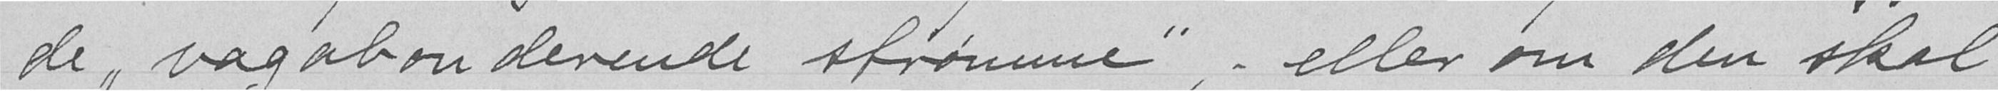de "vagabonderende strømme", eller om den skal
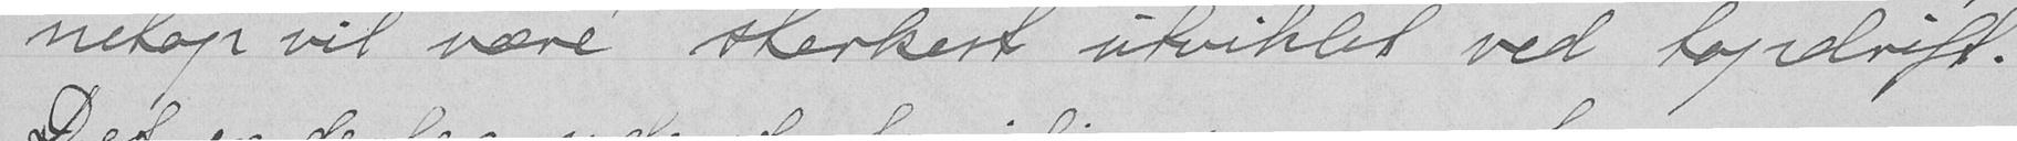netop vil være sterkest utviklet ved topdrift.
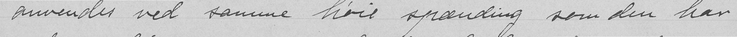anvendes ved samme høie spænding som den har
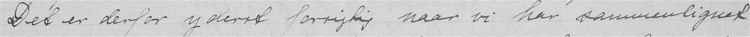Det er derfor yderst forsigtig naar vi har sammenlignet
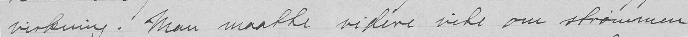virkning. Man maatte videre vite om strømmen
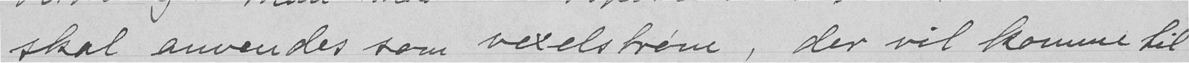skal anvendes som vexelstrøn, der vil komme til
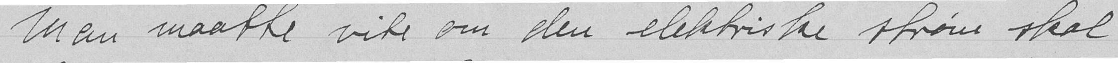Man maatte vite om den elektriske strøm skal
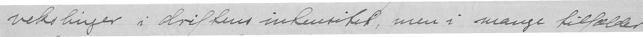vekslinger i driftens inhensitet, men i mange tilfælder
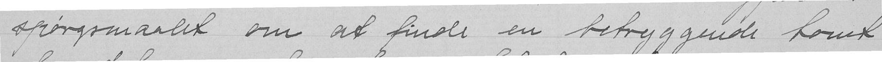spørgsmaalet om at finde en betryggende tomt
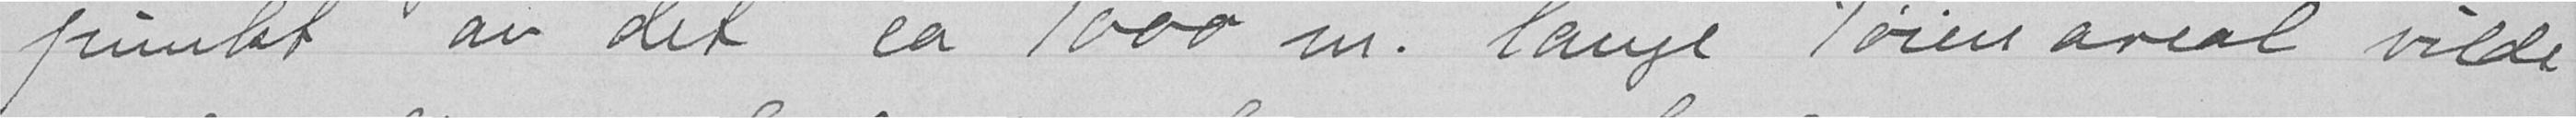punkt av det ca 1000 m. lange Tøienareal vilde
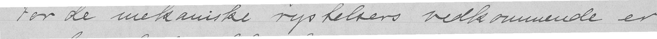For de mekaniske rystelsers vedkommende er
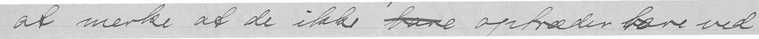at merke at de ikke bare optræder bare ved
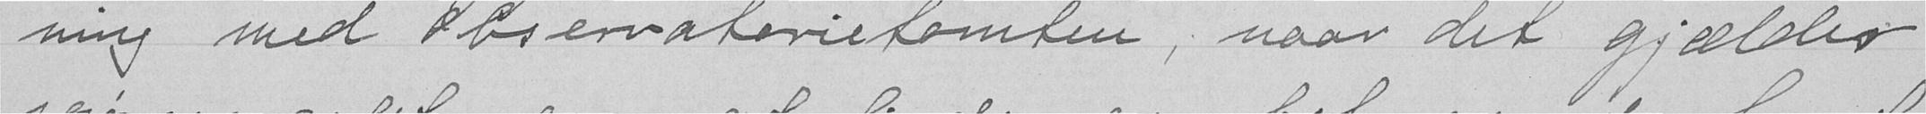ning med Observatorietomten, naar det gjælder
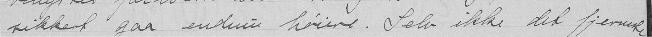sikkert gaa endnu høiere. Selv ikke det fjerneste
